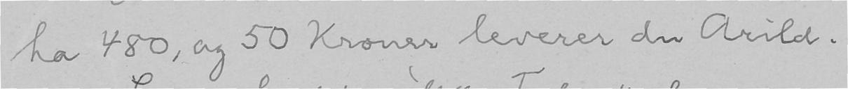ha 480, og 50 Kroner leverer du Arild.
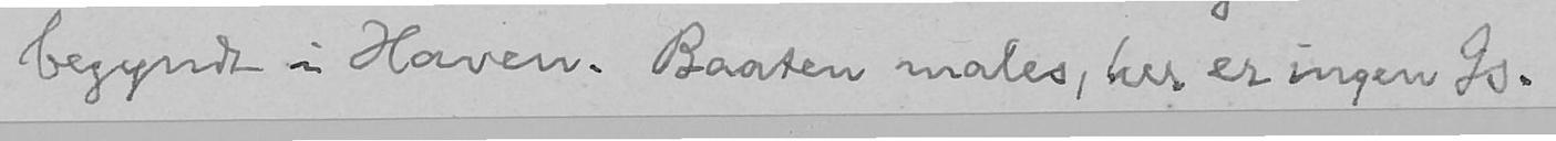begyndt i Haven. Baaten males, her er ingen Is.
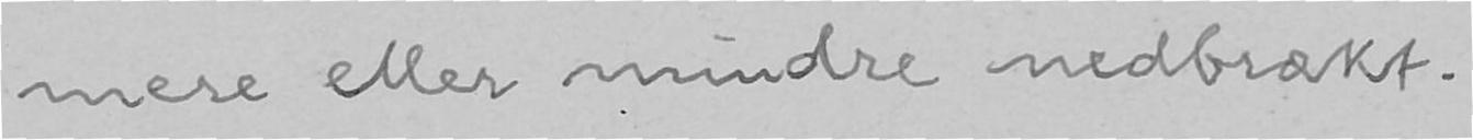mere eller mindre nedbrækt.
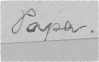Papa.
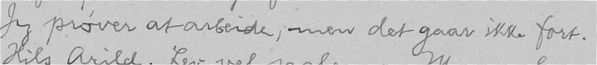Jeg prøver at arbeide, men det gaar ikke fort.
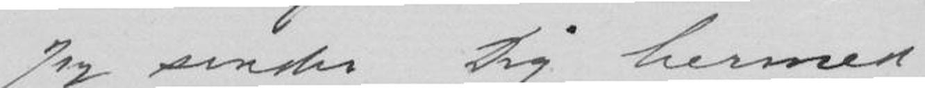Jeg sender Dig hermed
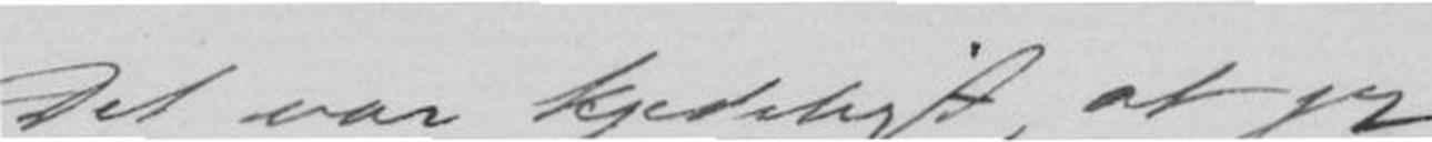Det var kjedeligt, at jeg
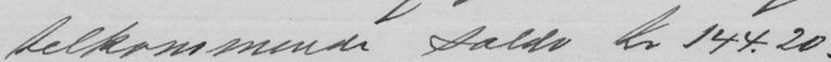tilkommende saldo kr 144.20.
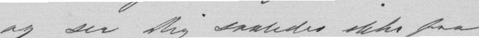og ser Dig saaledes ikke paa
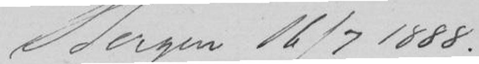Bergen 16/7 1888
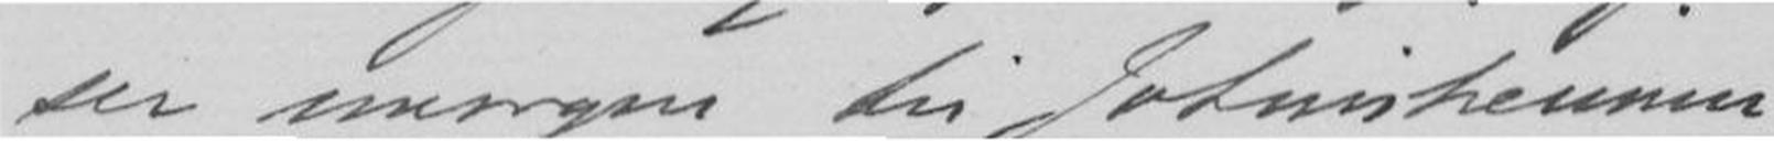ser imorgen til Jotunheimen
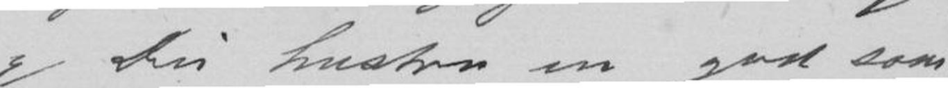og Din hustru en god som-
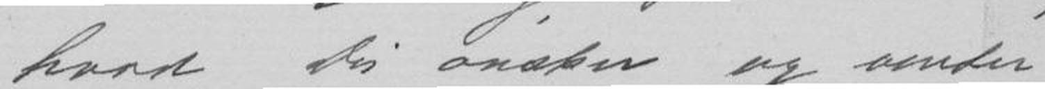hvad Du ønsker og venter
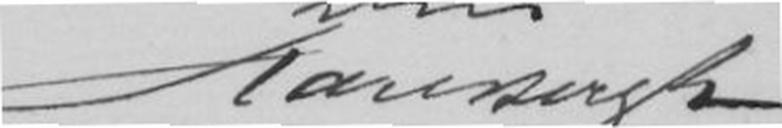Karl Bergh
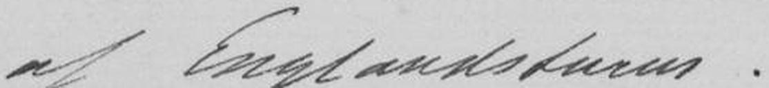af Englandsturen.
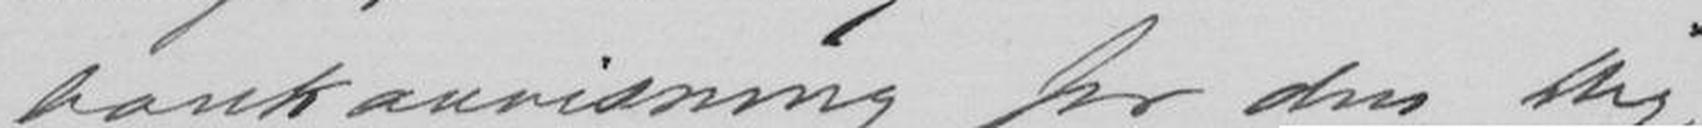bankanvisning for den Dig
